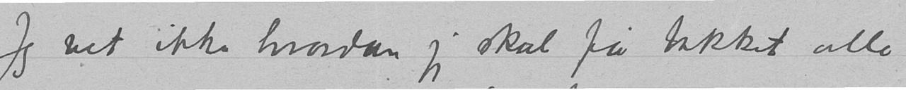Jeg vet ikke hvordan jeg skal få takket alle
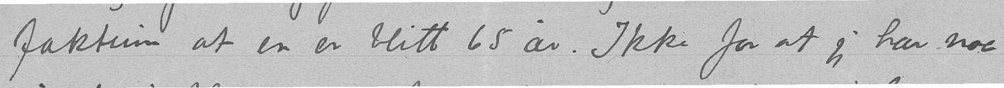faktum at en er blitt 65 år. Ikke for at jeg har noe
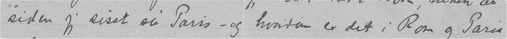siden jeg sisst så Paris – og hvordan er det i Rom og Paris
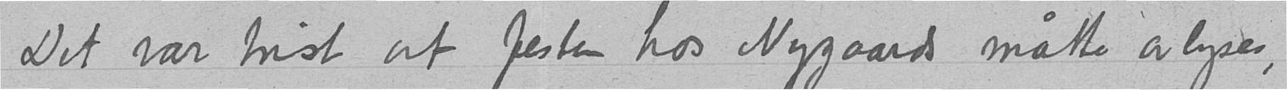Det var trist at festen hos Nygaards måtte avlyses,
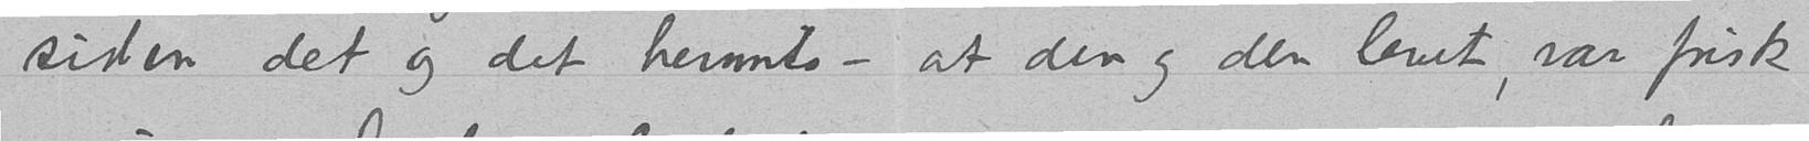siden det og det hennte – at den og den levet, var frisk
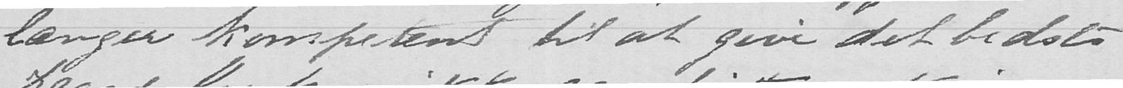længer kompetent til at give det bedste
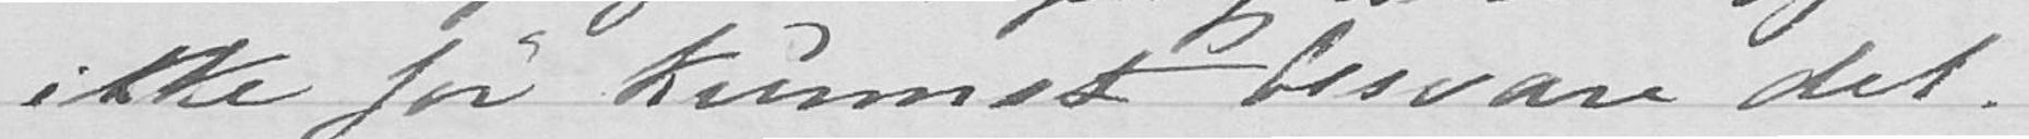ikke før kunnet besvare det.
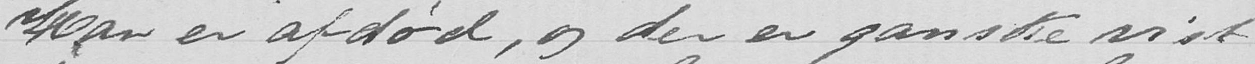Han er afdød, og der er ganske vist
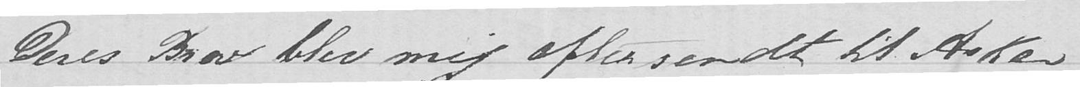Deres Brev blev mig eftersendt til Asker
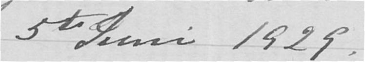5te Juni 1929
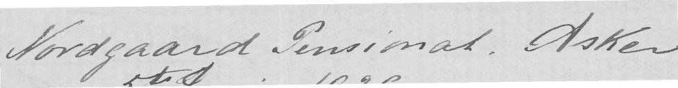Nordgaard Pensionat. Asker
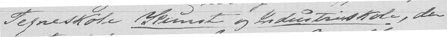Tegneskole Kunst og Industriskole, der
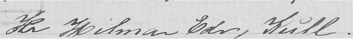Hr Hilmar Edv Kull.
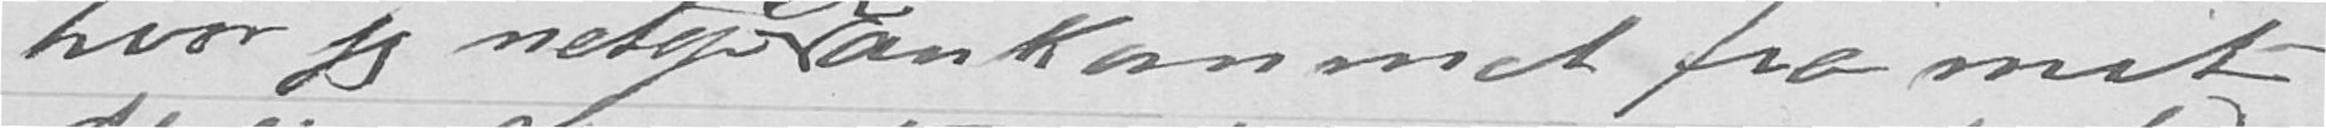hvor jeg netop er ankommet fra mit
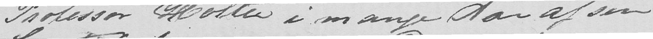Professor Holter i mange Aar af sin
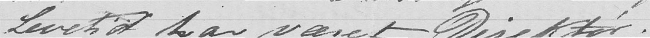Levetid har været Direktør.
